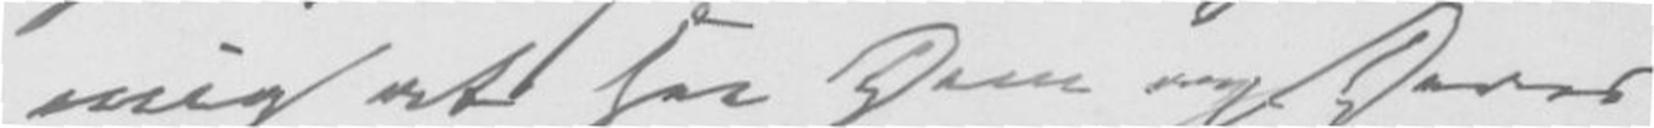mig at se Dem og Deres
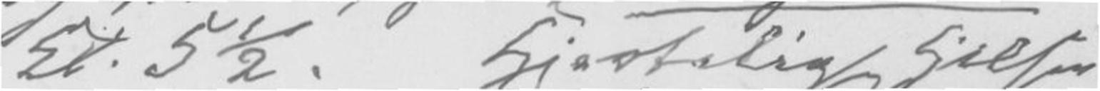kl. 5 1/2.Hjertelig Hilsen
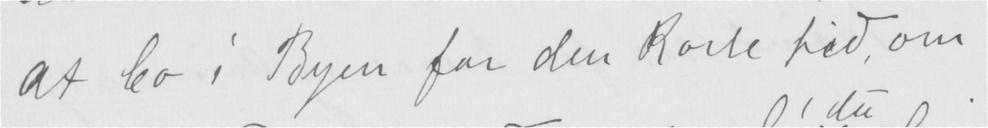at bo i Byen for den korte tid, om
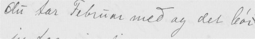du tar Februar med og det bør
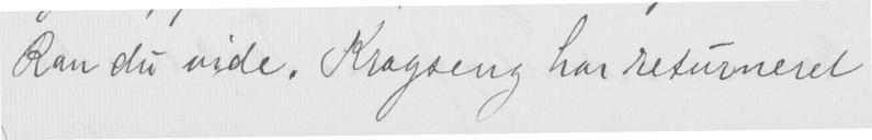kan du vide. Kragseng har returneret
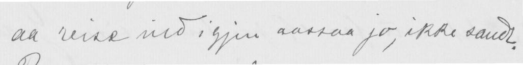aa reise ind igjen aassaa jo, ikke sandt.
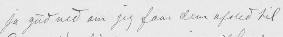ja gud ved om jeg faar dem afsted til
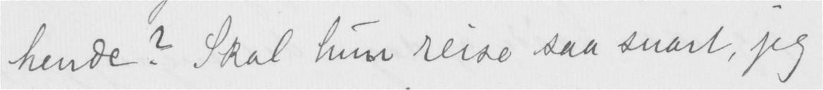hende? Skal hun reise saa snart, jeg
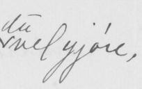vel gjøre,
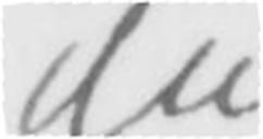du
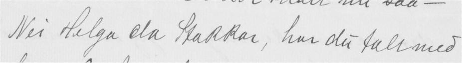Nei Helga da stakkar, har du talt med
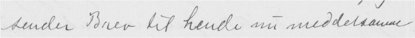sender Brev til hende nu med detsamme
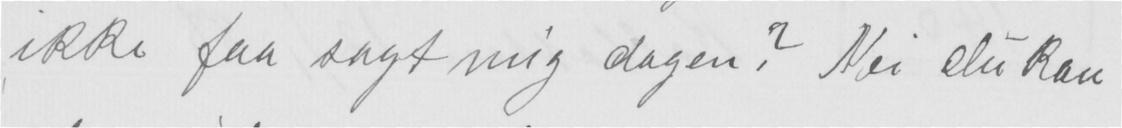ikke faa sagt mig dagen? Nei du kan
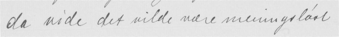da vide det vilde være meningsløst
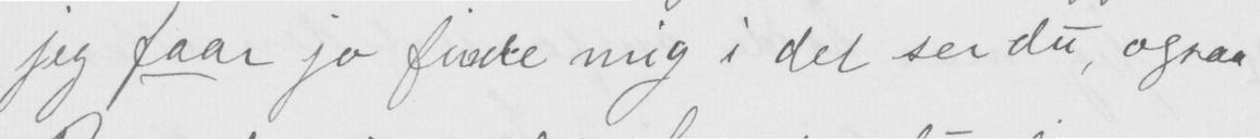jeg faar jo finde mig i det ser du, ogsaa
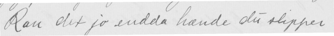kan det jo endda hænde du slipper
